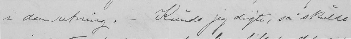i den retning. - Kunde jeg digte, så skulde
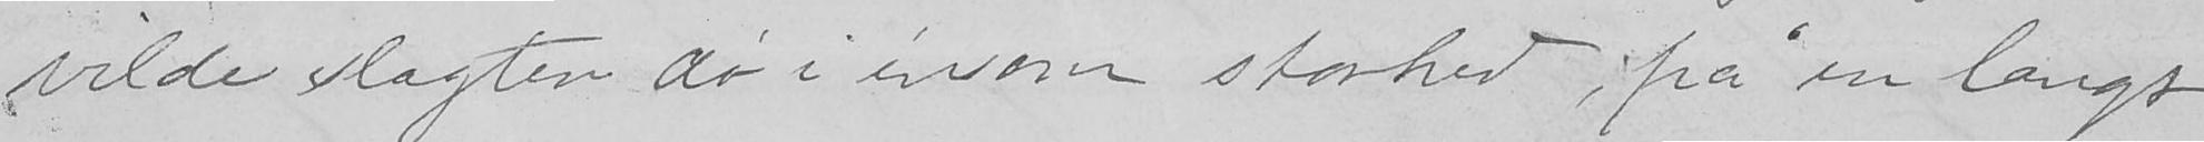vilde slægten dø i ensom storhed, på en langt
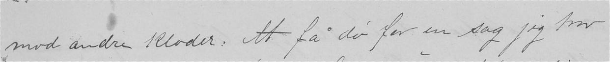mod andre kloder. At få dø for en sag jeg tror
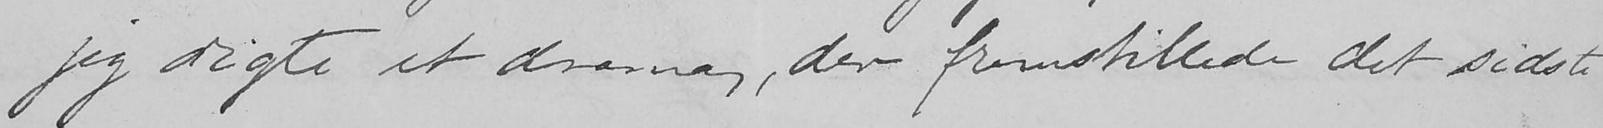jeg digte et drama, der fremstillede det sidste
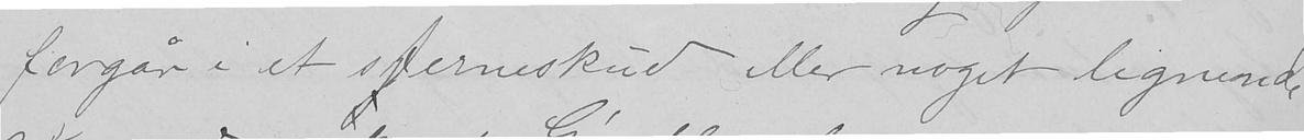forgår i et stjerneskud eller noget lignende.
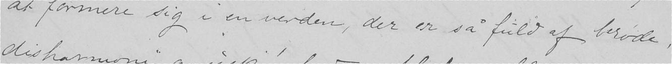at formere sig i en verden, der er så fuld af brøde,
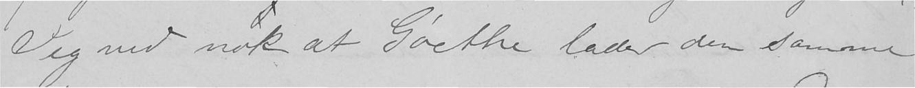Jeg ved nok at Goethe lader den samme
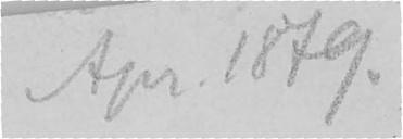Apr. 1879.
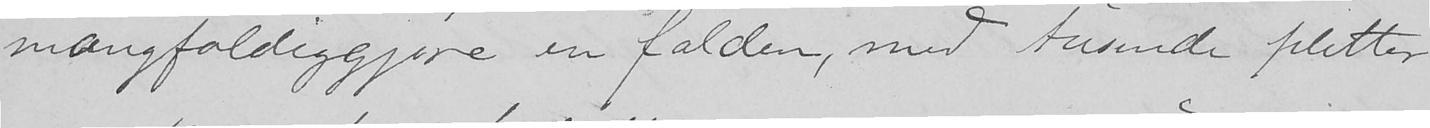mangfoldiggjøre en falden, med tusinde pletter
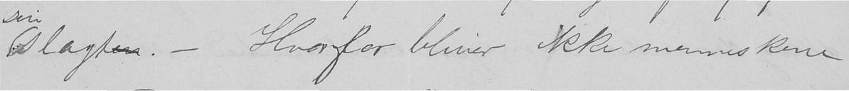slægten. - Hvorfor bliver ikke mennskene
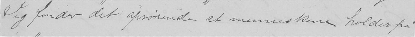Jeg finder det oprørende at menneskene holder på
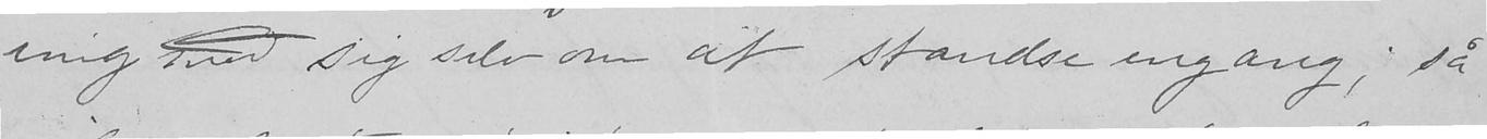enig med sig selv om at standse engang; så
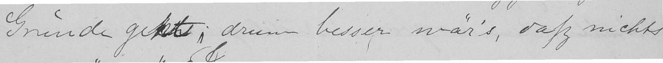Grunde geht; drum besser war's, dass nichts
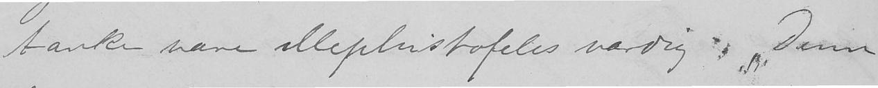tanke være Mephistofeles værdig; "Denn
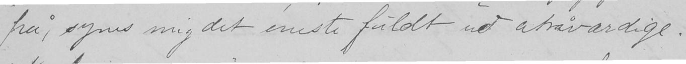på, synes mig det eneste fuldt ud atråværdige.
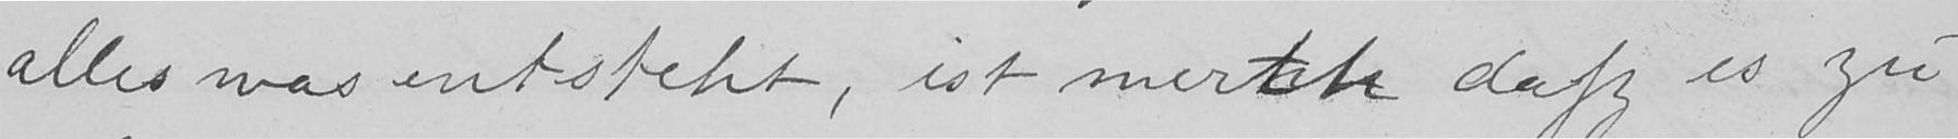alles was entsteht, ist werth dass es zu
